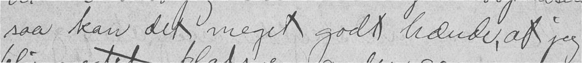saa kan det meget godt hænde, at jeg
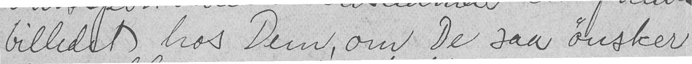billedet hos Dem, om De saa ønsker
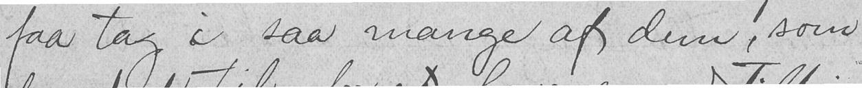faa tag i saa mange af dem, som
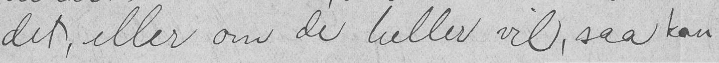det, eller om de heller vil, saa kan
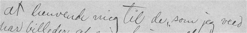at henvende mig til de, som jeg veed
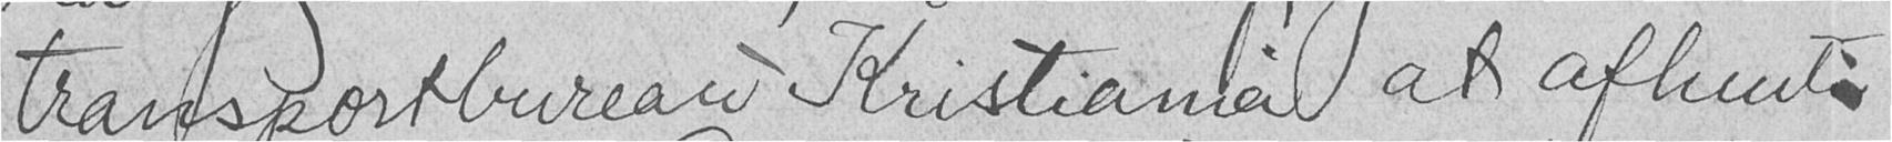transportbureau Kristiania at afhente
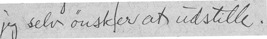jeg selv ønsker at udstille.
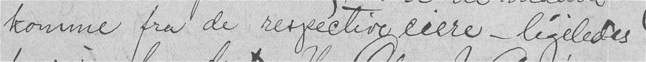komme fra de respective eiere - ligeledes
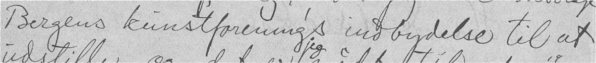Bergens kunstforening's indbydelse til at
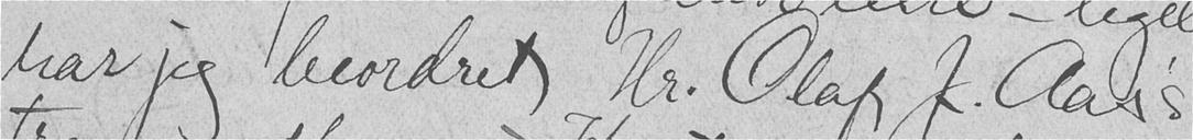har jeg beordret Hr. Olaf J. Aas's
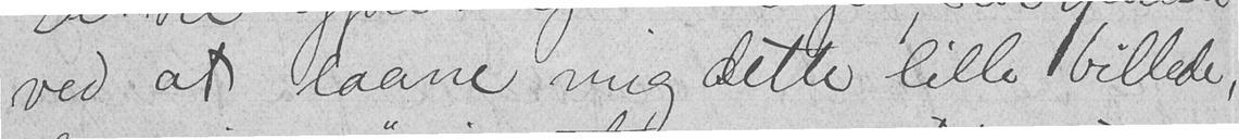ved at laane mig dette lille billede,
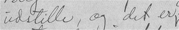udstille, og det er
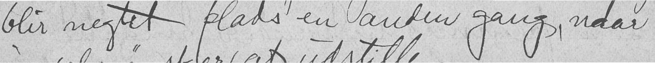blir negtet plads en anden gang, naar
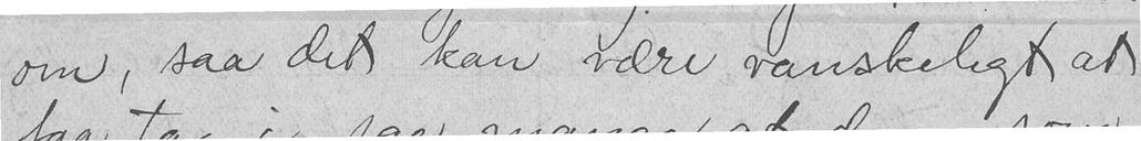om, saa dett kan være vanskeligt at
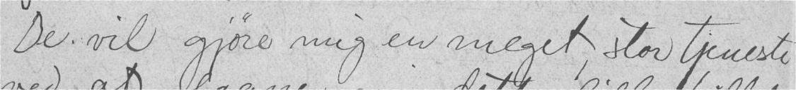De vil gjøre mig en meget, stor tjeneste
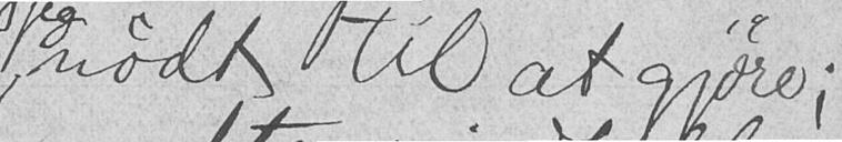nød til at gjøre;
